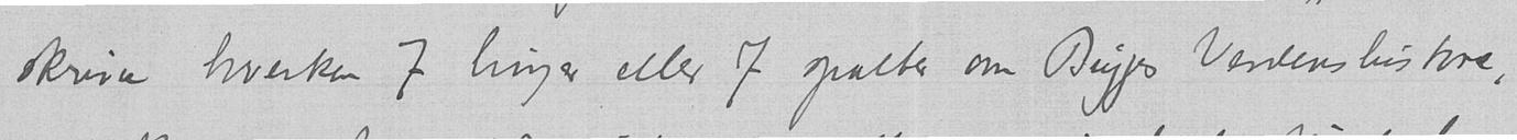skrive hverken 7 linjer eller 7 spalter om Bugges Verdenshistore,
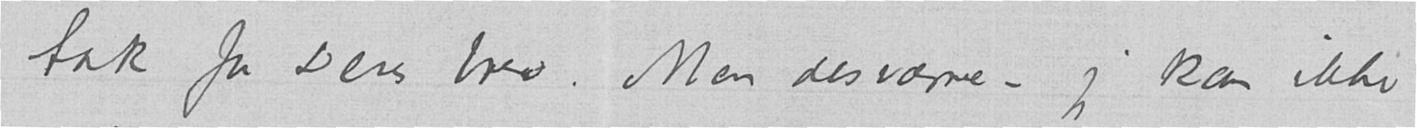tak for Deres brev. Men desværre – jeg kan ikke
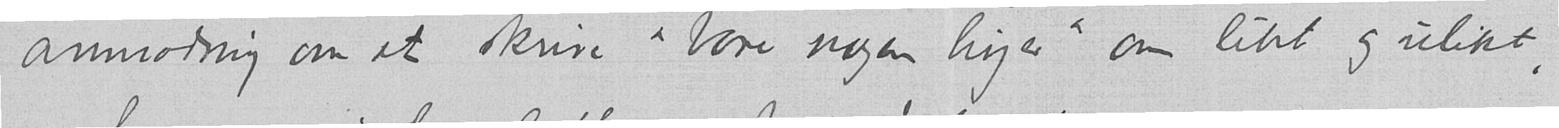anmodning om at skrive "bare nogen linjer" om likt og ulikt,
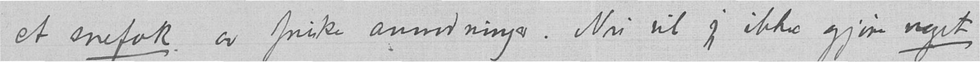et snefok av friske anmodninger. Nu vil jeg ikke gjøre noget,
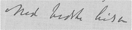Med bedste hilsen
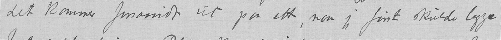det kommer forsaavidt ut paa ett, naar jeg først skulde legge
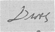Deres
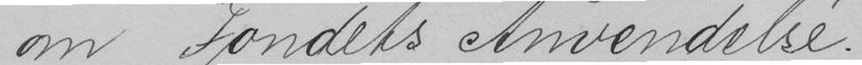om Fondets Anvendelse.
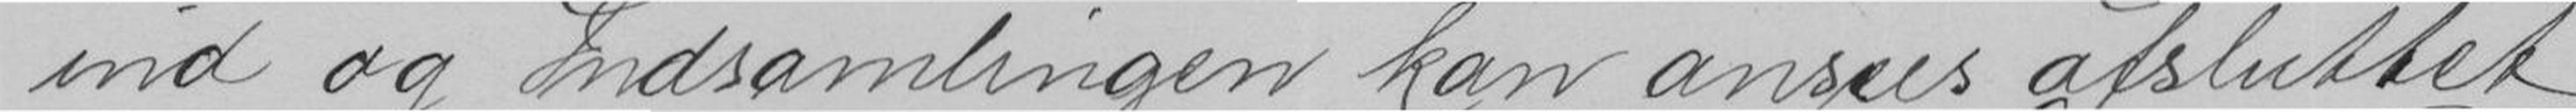ind og Indsamlingen kan ansees afsluttet,
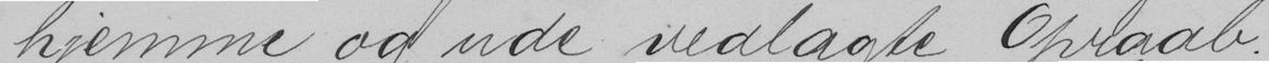hjemme og ude vedlagte Opraab.
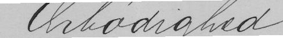I Ærbødighed
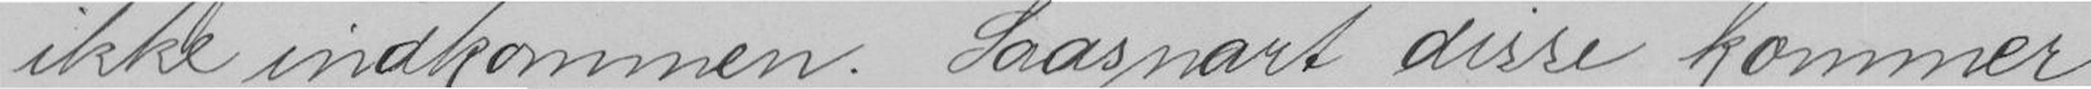ikke indkommen. Saasnart disse kommer
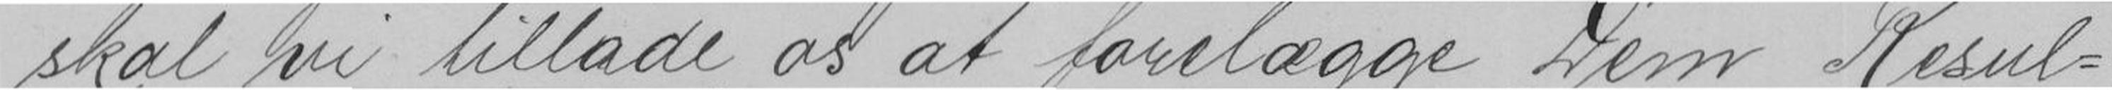skal vi tillade os at forelægge Dem Resul-
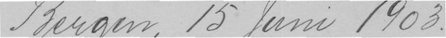Bergen, 15 Juni 1903
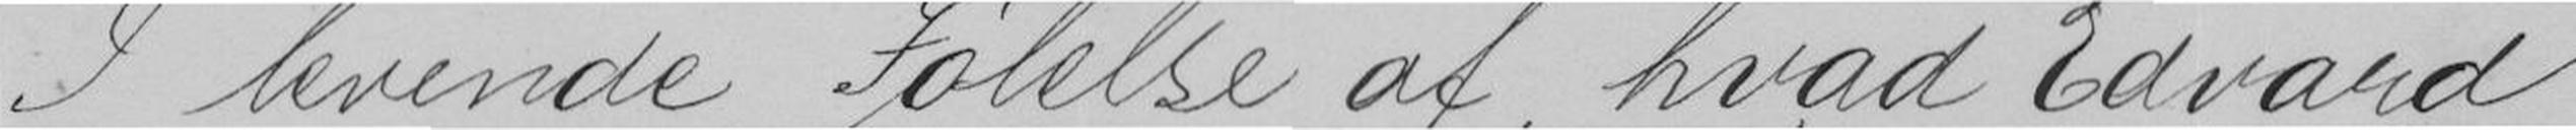I levende Følelse af, hvad Edvard
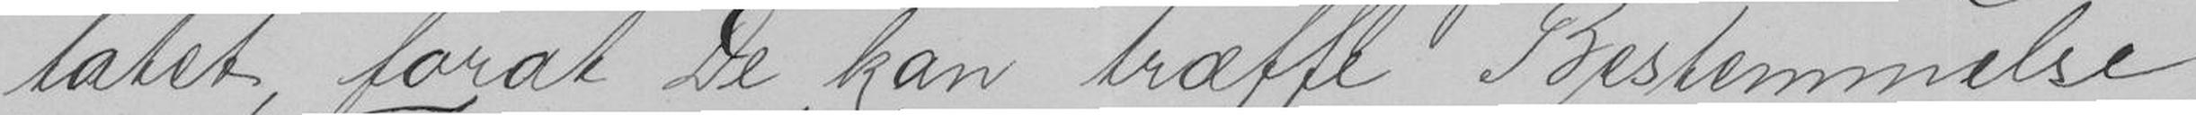tatet, forat De kan træffe Bestemnmelse
